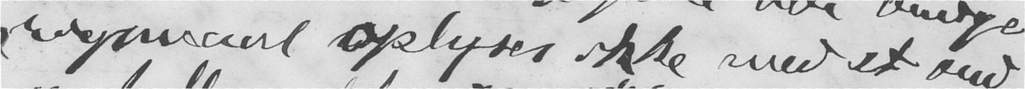rigsmaal oplyses ikke med et ord,
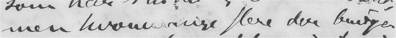men hvormange flere der bruger
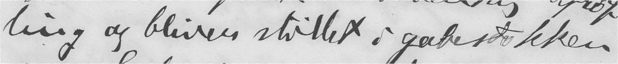ling og bliver stillet i gabestokken
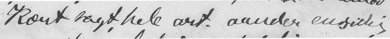Kort sagt, hele art. aander ensidig
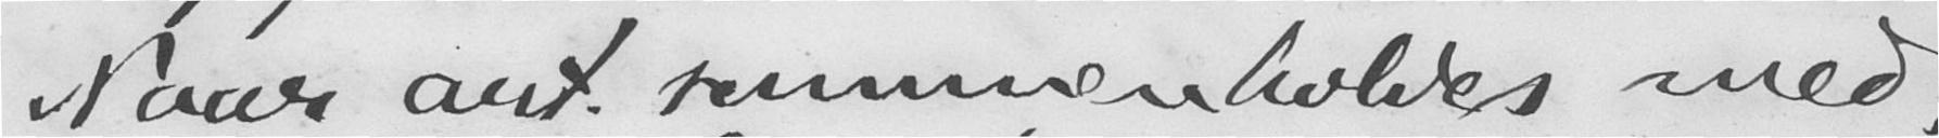Naar art. sammenholdes med,
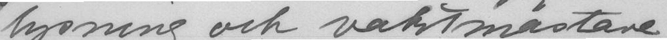lysning och vaktmästare,
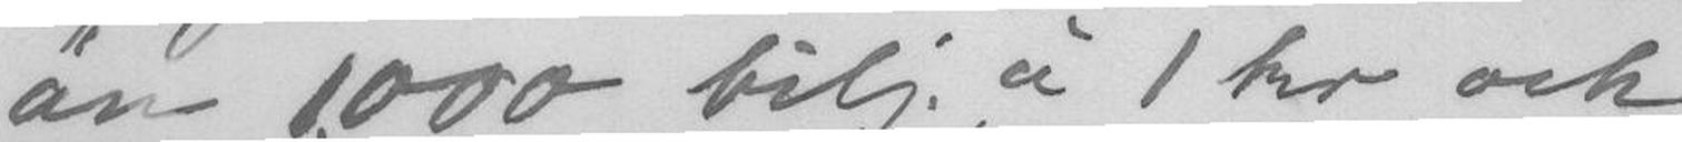är - 1 000 blij. á 1 kr. och
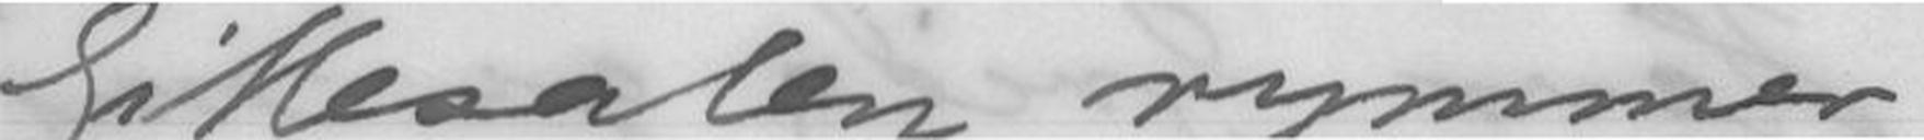Spillesalen rymmer
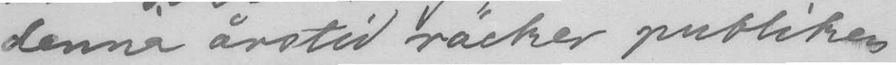denna årstid ræcker publiken
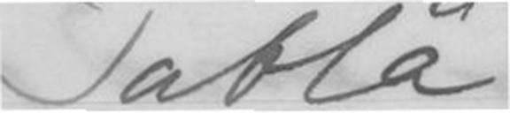Tablå
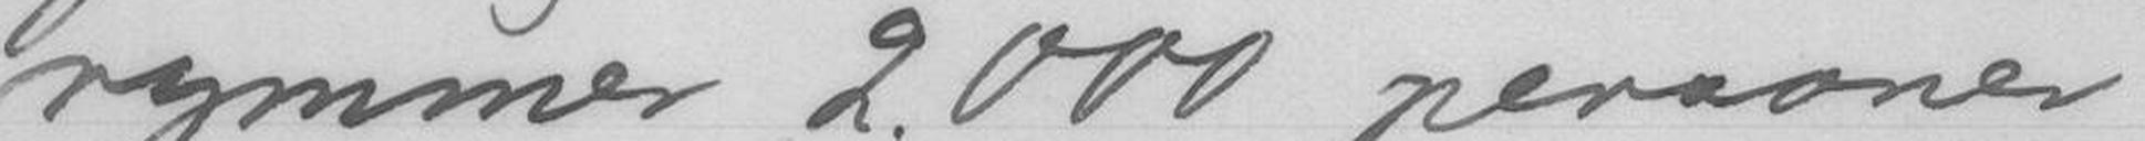rymmer 2.000 personer
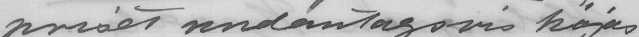prisen undantagsvis høgas
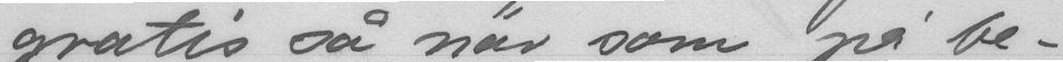gratis så när som på be-
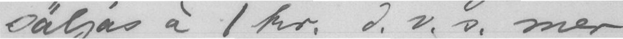säljas á 1 kr. d.v.s. mer
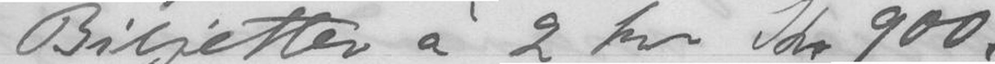Biljetter á 2 kr Kr. 900.
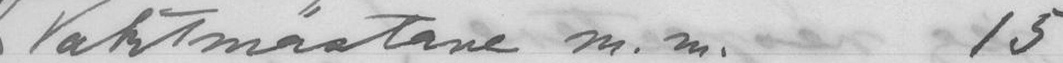Vaktmæstane m.m. 15
